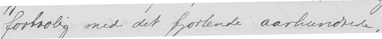fortrolig med det fjortende aarhundrede,
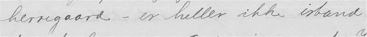herregaard - er heller ikke istand
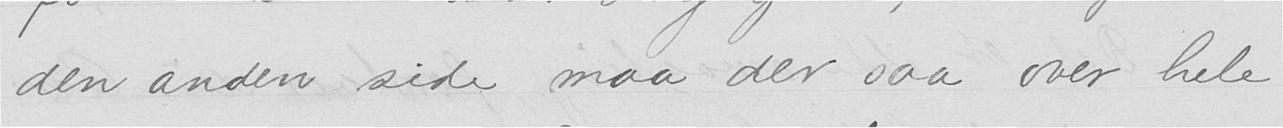den anden side maa der saa over hele
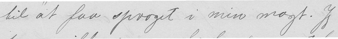til at faa sproget i min magt. Jeg
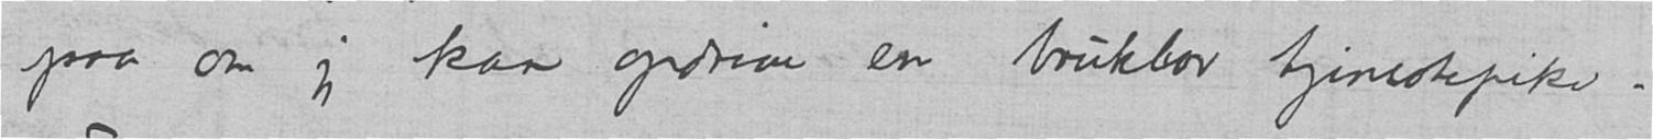paa om jeg kan opdrive en brukbar tjenestepike.
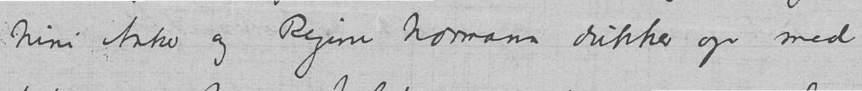Nini Anker og Regine Normann dukker op med
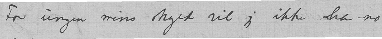For ungen mins skyld vil jeg ikke ha no
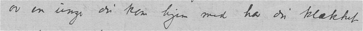av en unge du kom hjem med har du klækket
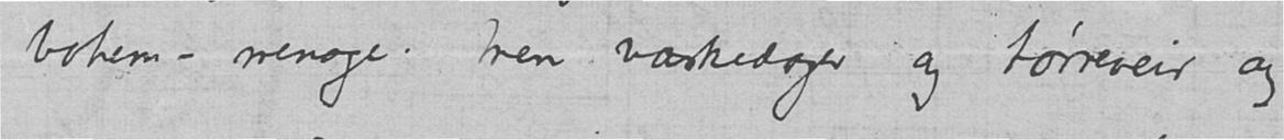bohem-menage. Men vaskedager og tørreveir og
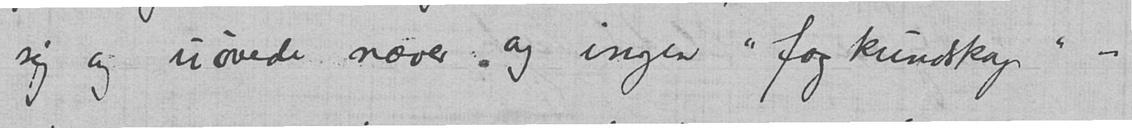sig og uøvede næver - og ingen "fagkundskap" -
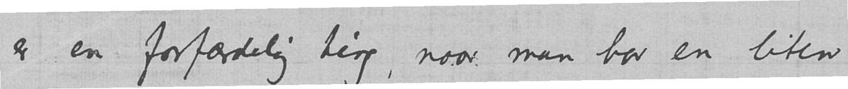er en forfærdelig ting, naar man har en liten
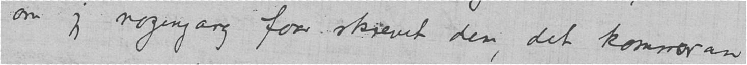om jeg nogengang faar skrevet den, det kommer an
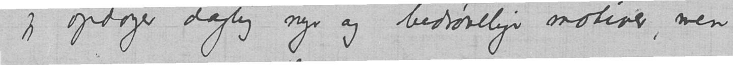jeg opdager daglig nye og bedrøvelige motiver, men
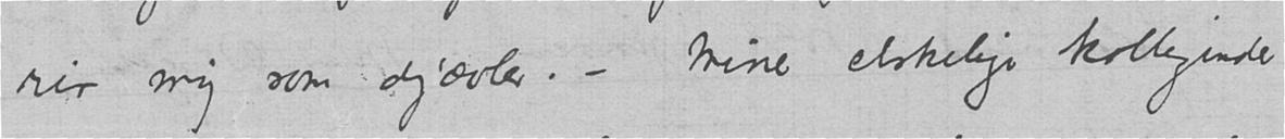rir mig som djævler. – Mine elskelige kolleginder
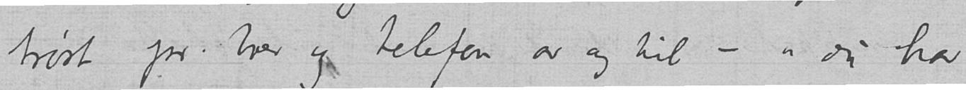trøst pr. brev og telefon av og til – "du har
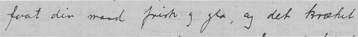faat din mand frisk og gla, og det kræket
